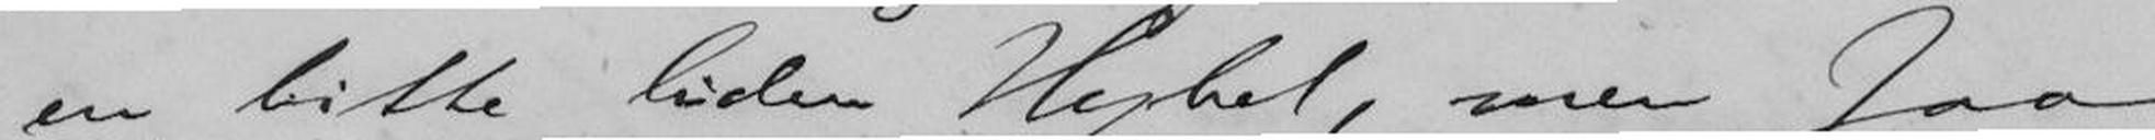en bitte liden Hybel, men saa
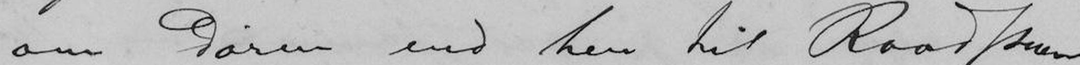om Døren end hen til Raadstuen
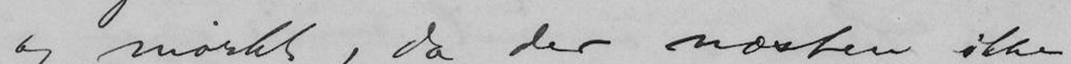og mørkt, da der næsten ikke
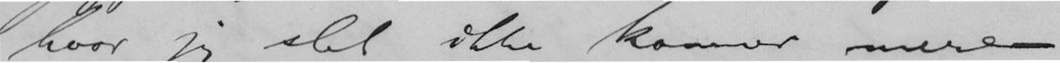hvor jeg slet ikke kommer mere
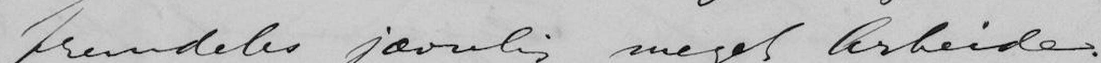fremdeles jævnlig meget Arbeide.
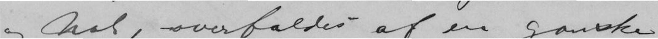og Nat, overfaldes af en ganske
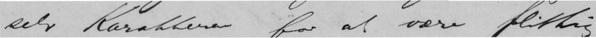selv Karakteren for at være flittig
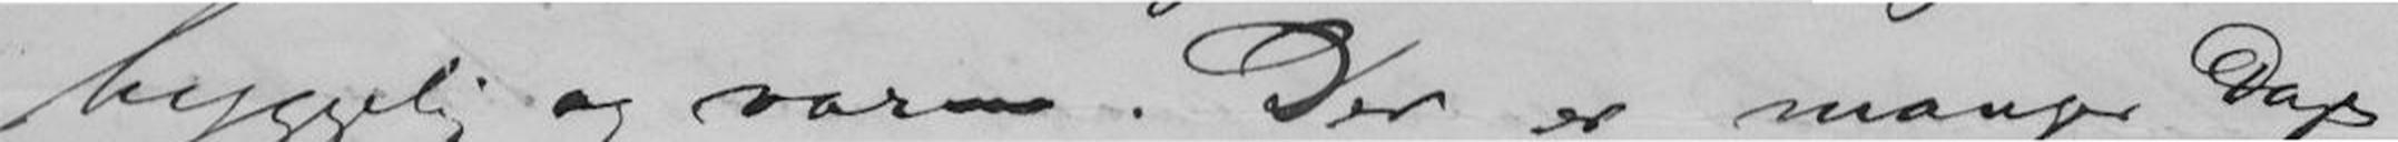hyggelig og varm. Der er mange Dage
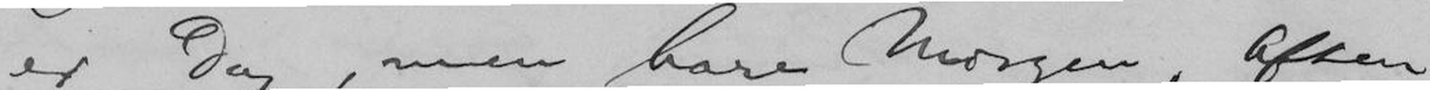er Dag, men bare Morgen, Aften
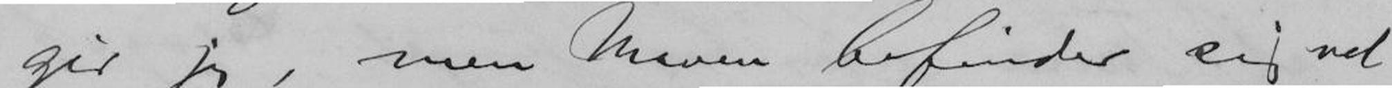gir jeg, men Maven befinder sig vel
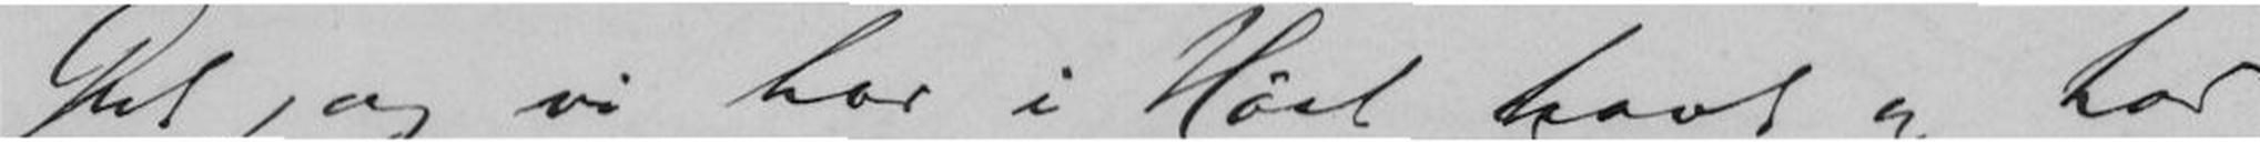Gut, og vi har i Høst havt og har
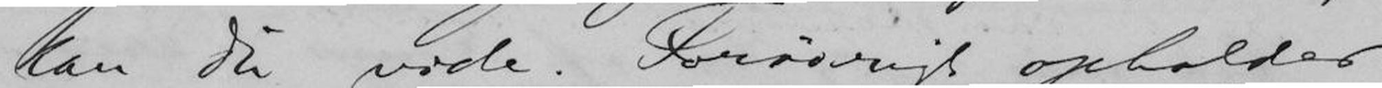kan du vide. Forøvrigt opholder
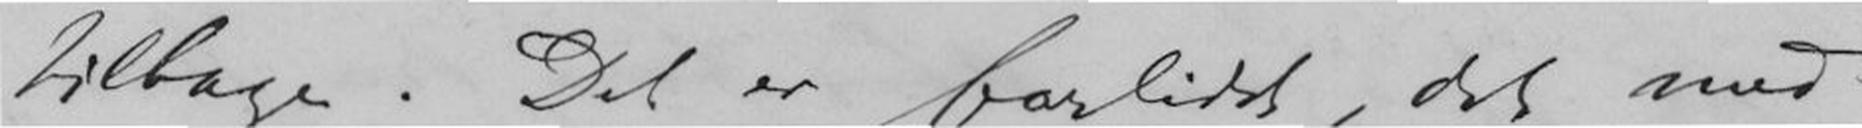tilbage. Det er forlidet, det med-
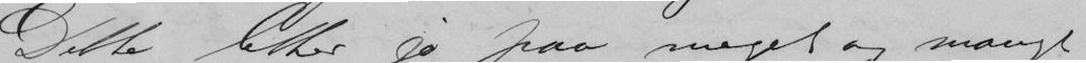Dette letter jo paa meget og mangt
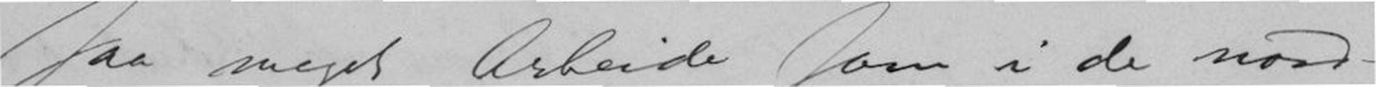saa meget Arbeide som i de nord-
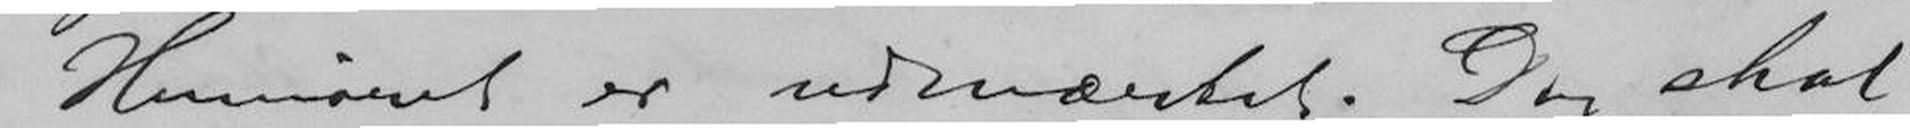Humøret er udmærket. Dog skal
